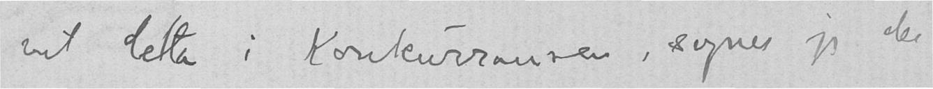vil delta i Konkurransen, synes jeg der
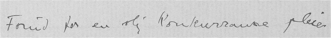Forud for en slig Konkurranse pleier
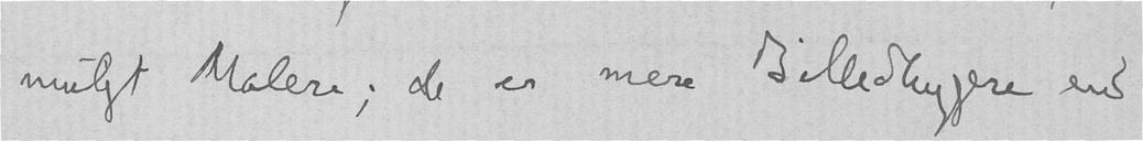mulgt Malere; de er mere Billedhuggere end
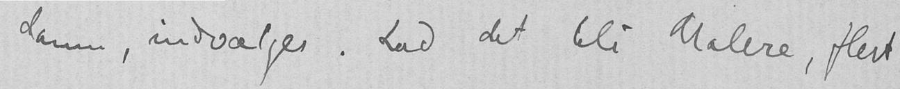danne, indvælges. Lad det bli Malere, flest
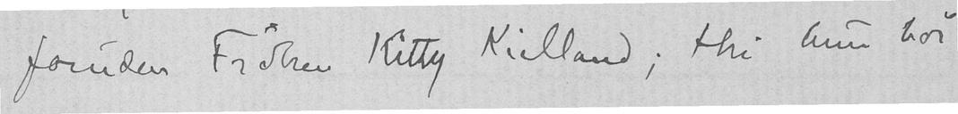foruden Frøken Kitty Kielland; thi hun bør
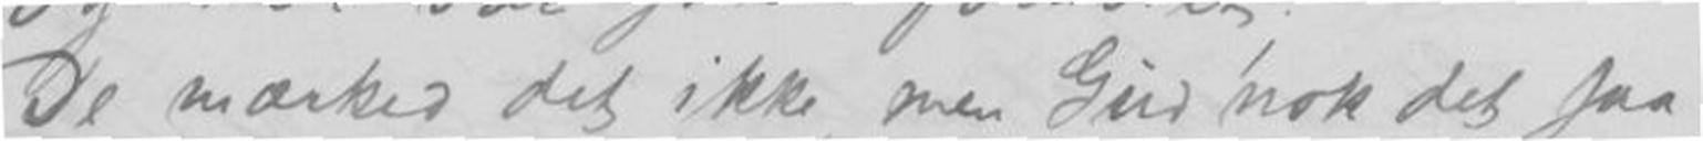De mærked det ikke, men Gud nok det saa,
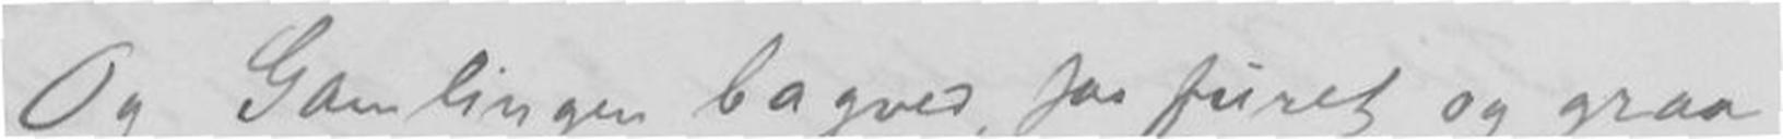Og Gamlingen bagved, saa furet og graa
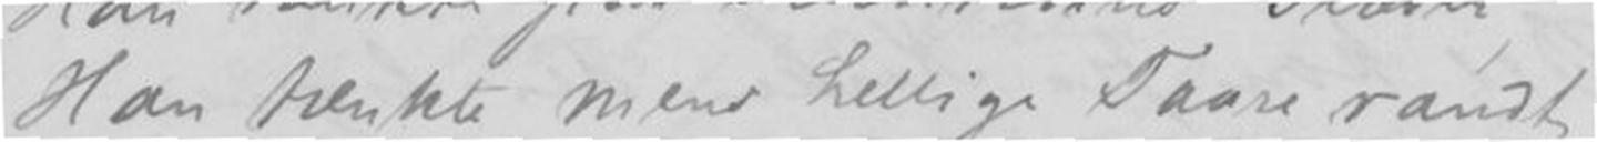Han tænkte, mens hellige Taare randt
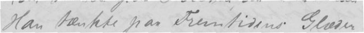Han tænkte paa Fremtidens Glæder,
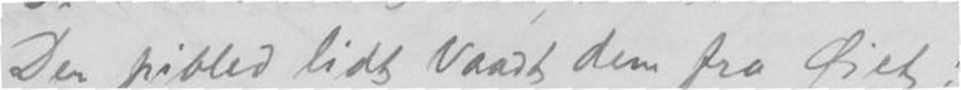Der pibled lidt Vaadt dem fra Øiet:
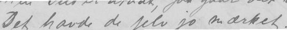Det havde de selv jo mærket. -
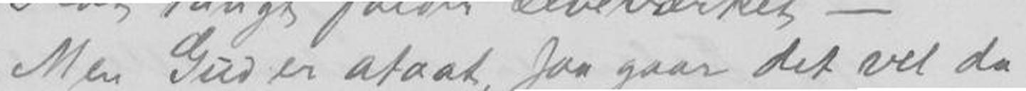Men Gud er ataat, saa gaar det vel da
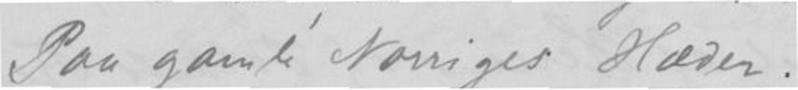Paa gamle Noriges Hæder.
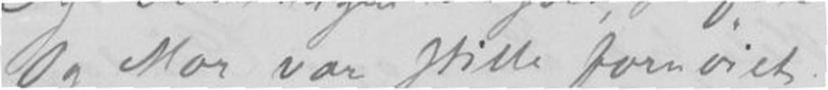Og Mor var stille fornøiet.
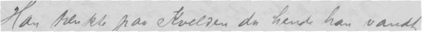Han tænkte paa Kvelden, da hende han vandt,
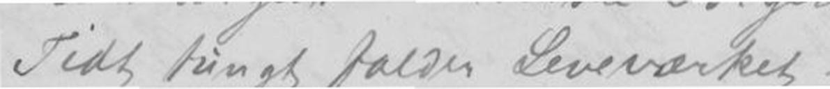Tidt tungt falder Leveværket -
